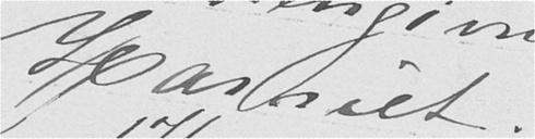Harriet.
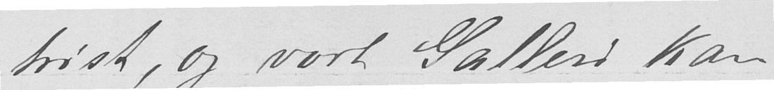trist, og vort Galleri kan
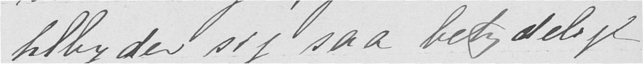tilbyder sig saa betydeligt
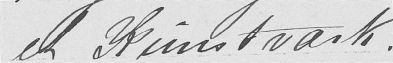et Kunstværk.
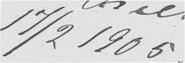17/2 1905.
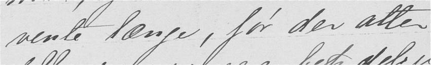vente længe, før der atter
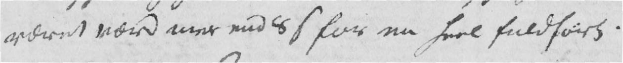været værd mer end 8 s for en heel fuldført.
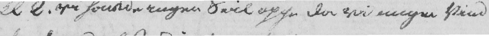Kl 2. vi havde ingen Seil oppe da vi ingen Vind
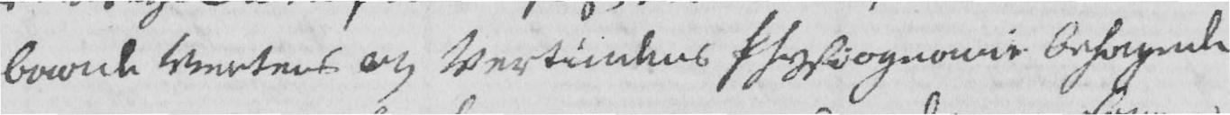baade Vertens og Vertindens Pfysiognomie behagede
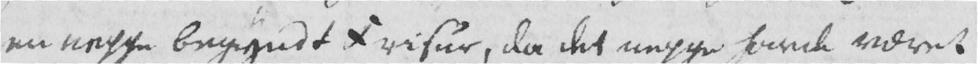en neppe begyndt Friseur, da det neppe havde været
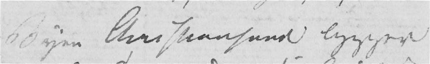Byen Christiansund ligger
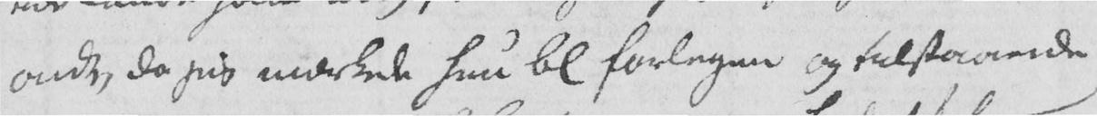ondt, da jeg mærkede hun bl forlegen og tilstaaende
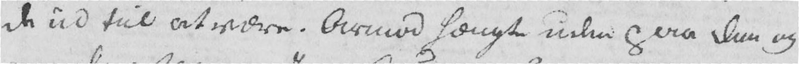de ud til at være. Armod hængte uden paa dem og
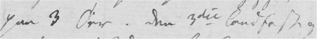paa 3 Øer. Den 3die landfast og
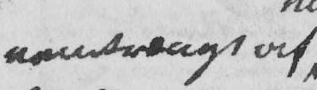nemtrængt af
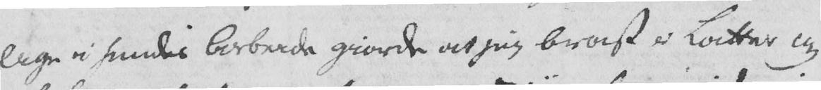lige i hendes Arbeide giorde at jeg brast i Latter og
